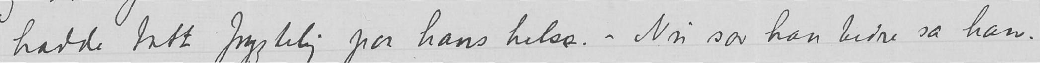hadde tatt frygtelig paa hans helse. – Nu sov han bedre sa han.
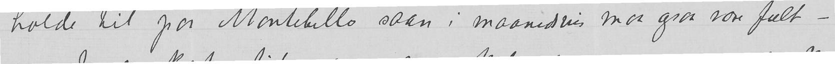holde til paa Montebello saan i maanedsvis maa ogsaa være fælt –
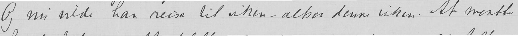Og nu vilde han reise til uken – altsaa denne uken. At maatte
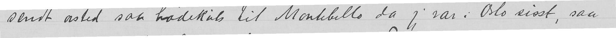sendt avsted saa hodekåls til Montebello da jeg var i Oslo sisst, saa
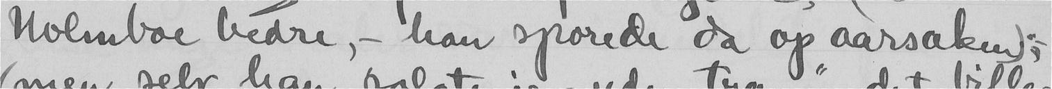Holmboe bedre, - han sporede da op aarsaken);
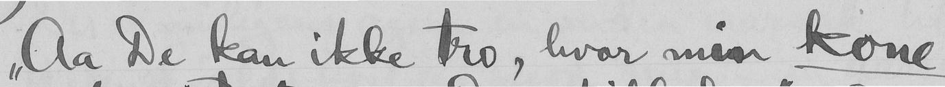"Aa De kan ikke tro, hvor min kone
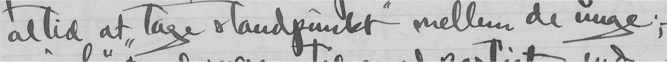altid at "tage standpunkt" mellem de unge;
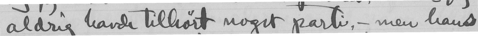aldrig havde tilhørt noget parti, - men hans
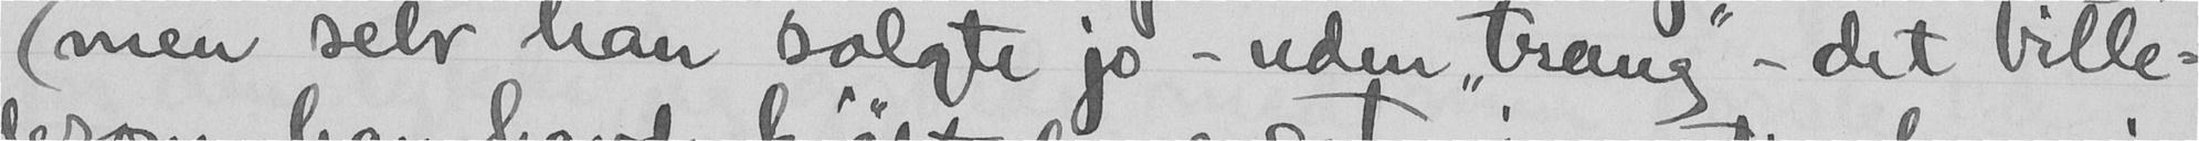(men selv han solgte jo - uden "trang" - det bille-
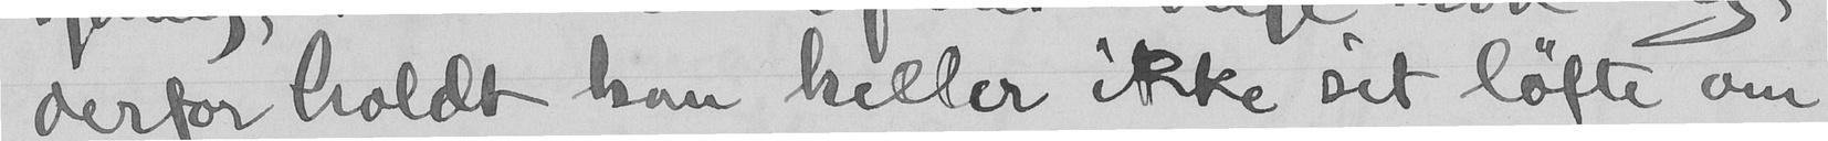derfor holdt hun heller ikke sit løfte om
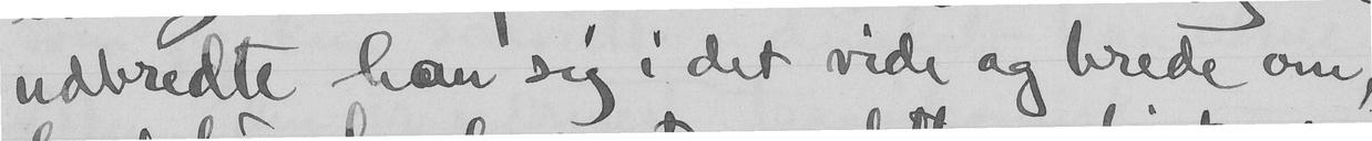udbredte han sig i det vide og brede om,
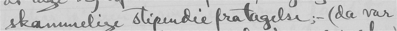skammelige stipendie fratagelse; - (da var
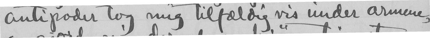antipoder tog mig tilfældig vis under armene,
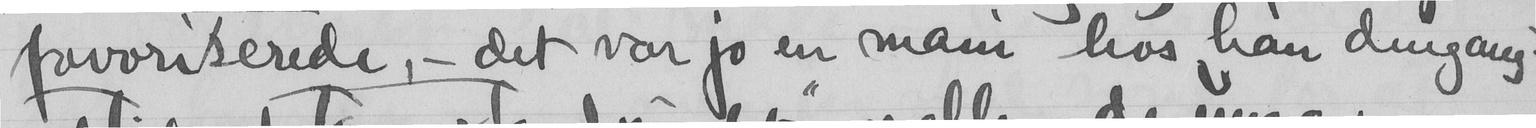favoriserede, - det var jo en mani hos han dengang
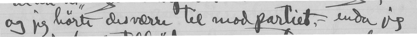og jeg hørte desværre til modpartiet, - enda jeg
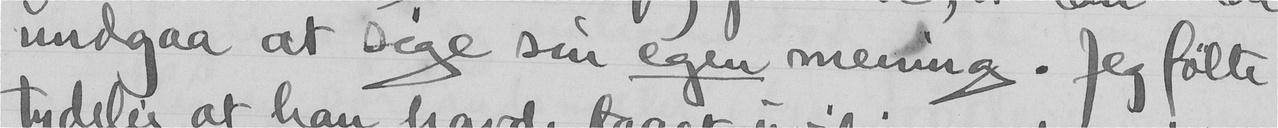undgaa at sige sin egen mening. Jeg følte
